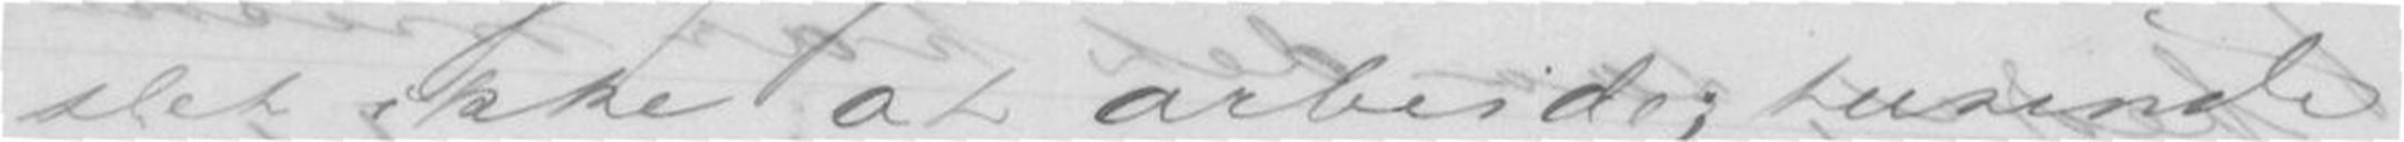slet ikke at arbeide; tusinde
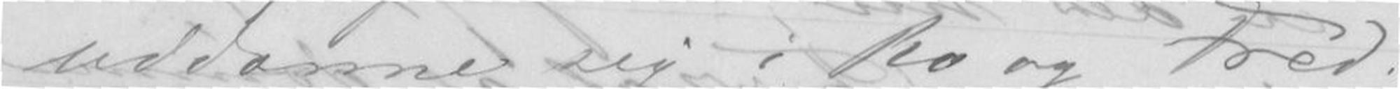uddanne sig i Ro og Fred.
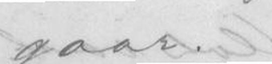gaar.
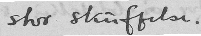stor skuffelse.
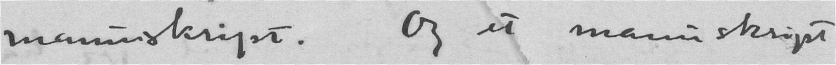manuskript. Og et manuskript
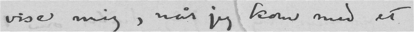vise mig, når jeg kom med et
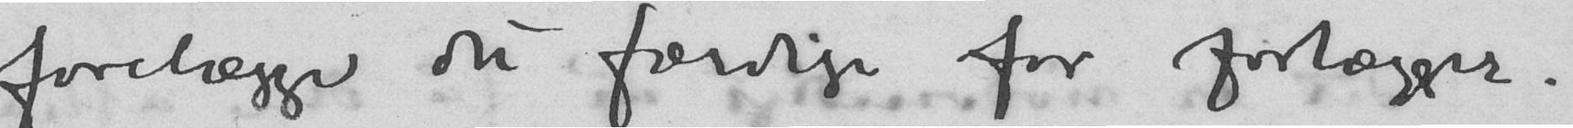forelægge det færdige for forlægger.
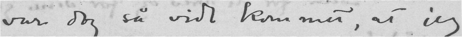var dog så vidt kommet, at jeg
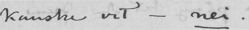kanske vet - nei.
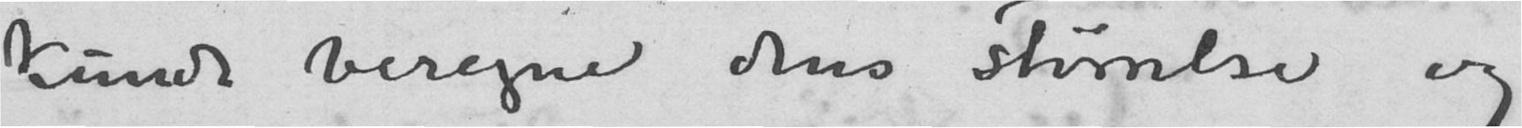kunde beregne dens størrelse og
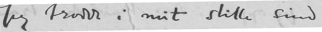Jeg trodde i mit stille sind
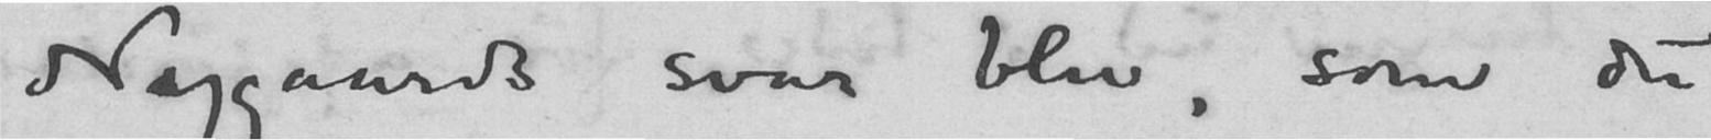Nygaards svar blev, som du
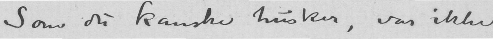Som du kanske husker, var ikke
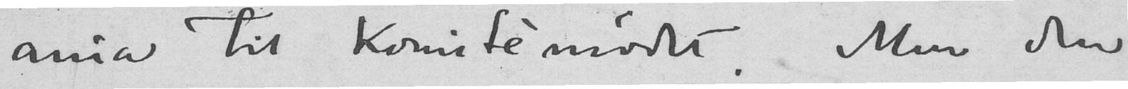ania til komitémødet. Men den
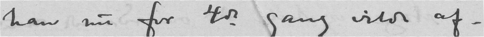han nu for 4de gang vilde af-
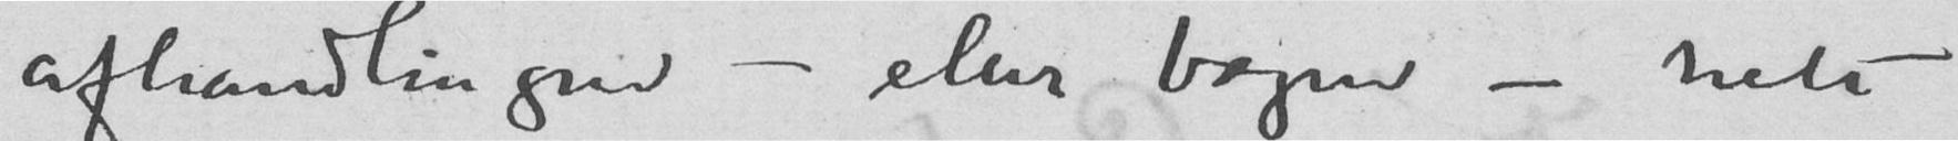afhandlingen - eller bogen - helt
